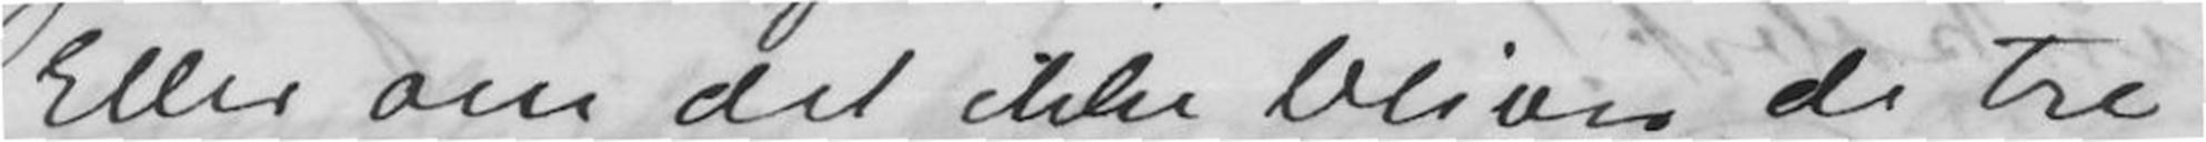Eller om det ikke bliver de tre
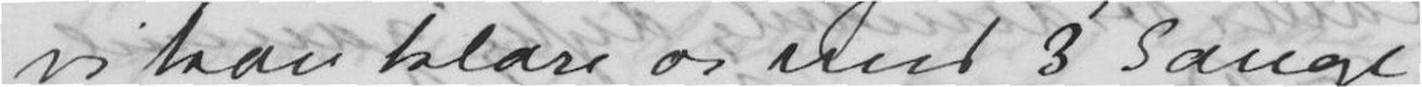vi kan klare os med 3 Gange
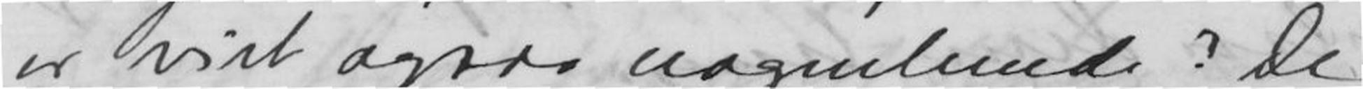er vist ogsaa nogenlunde? De
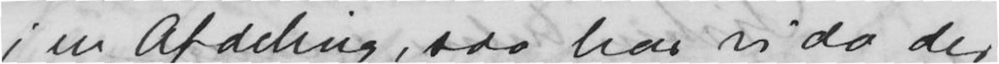i en Afdeling, saa har vi da der
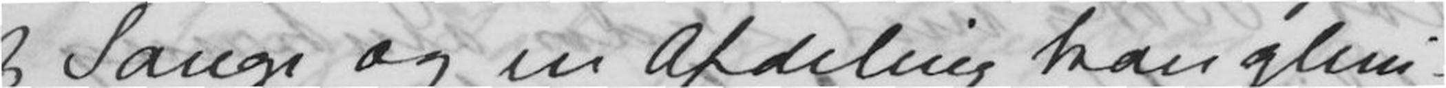3 Sange og en Afdeling kan glim-
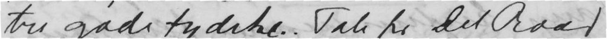tre gode tydske. Tak for Det Raad
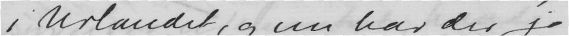i Udlandet, og nu har der jo
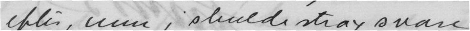efter, men j skulde strax svare
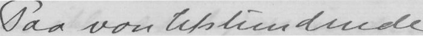Paa vore tilstundende
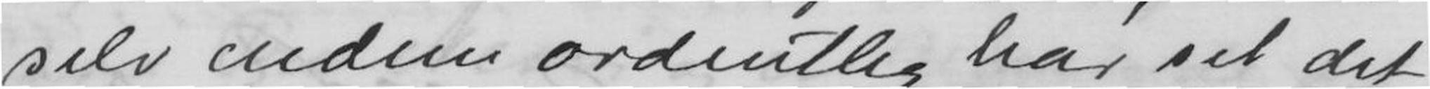selv endnu ordentlig har set det
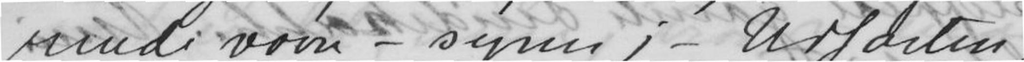rende vaere - synes j - Udfarten,
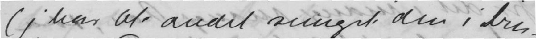(j har bl. andet sunget den i Dres-
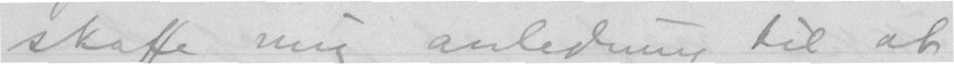skaffe mig anledning til at
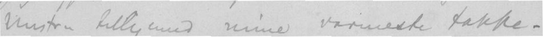hustru tilligemed mine varmeste takke-
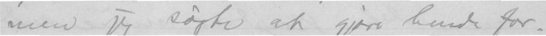men jeg søgte at gjøre hende for-
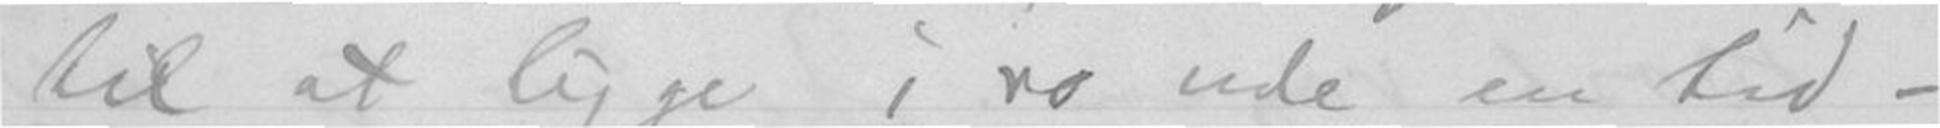til at ligge i ro ude en tid -
